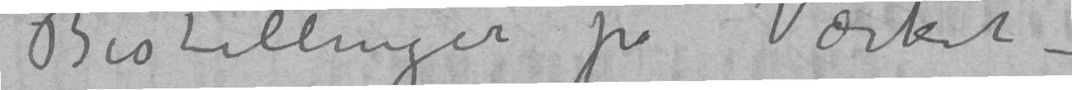Bestillinger på Værket –
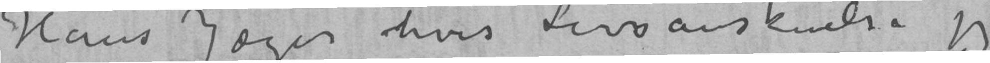Hans Jæger hvis Livsanskuelse jeg
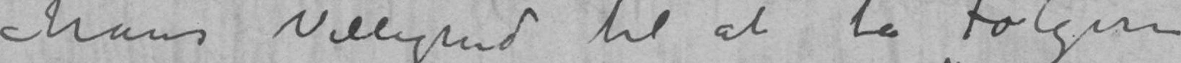– hans Villighed til at ta Følgen
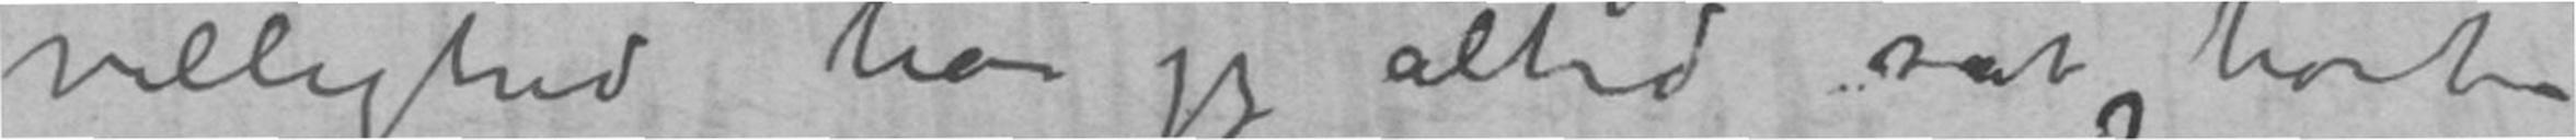villighed har jeg altid sat høit
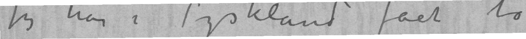Jeg har i Tyskland fået to
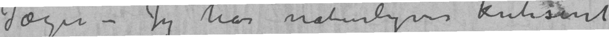Jæger – Jeg har naturligvis kritiseret
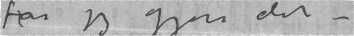får jeg gjøre det –
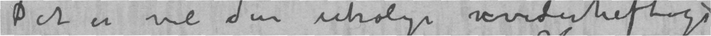Det er vel den utrolige vederheftige
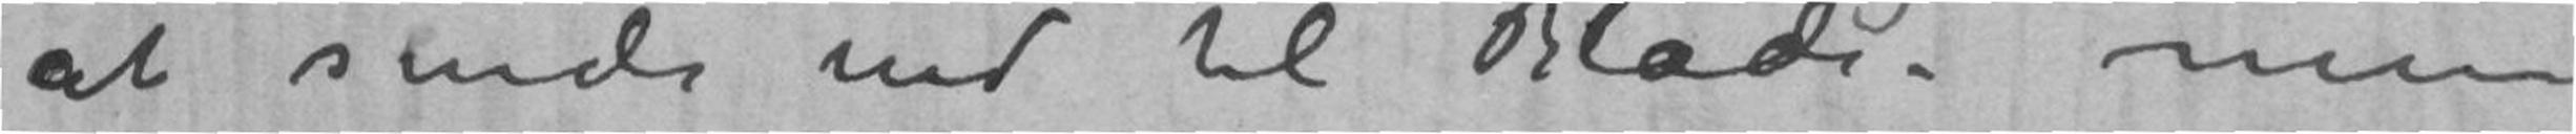at sende ind til Blade men
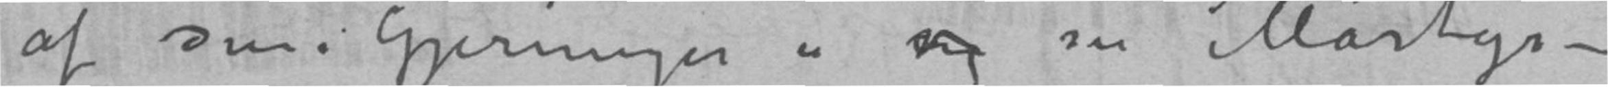af sine Gjerningerog sin «Martyr-
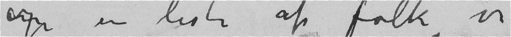op en liste af folk vi
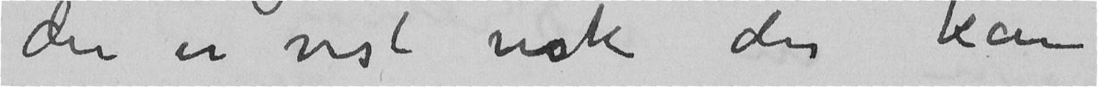der er vist nok der kan
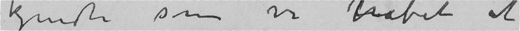kjendte som vi håbet at
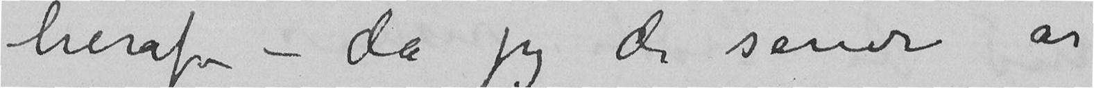heraf – da jeg de senere år
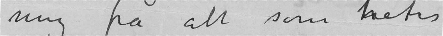mig fra alt som heter
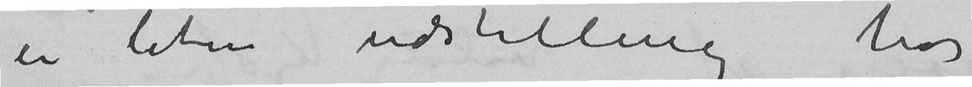en liten udstilling hos
